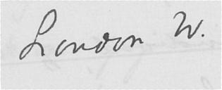London W.
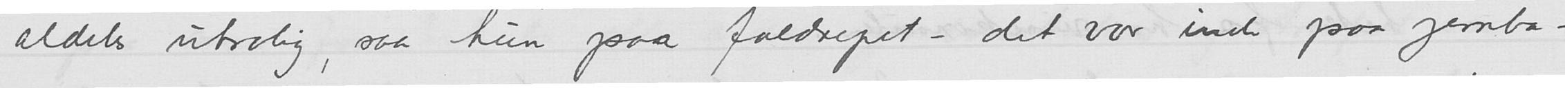aldeles utrolig, saa hun paa faldrepet — det var inde paa jernba-
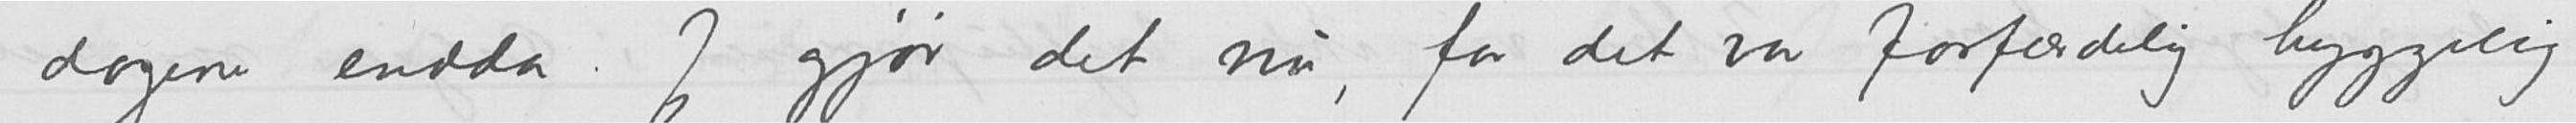dagene endda. Jeg gjør det nu, for det var forfærdelig hyggelig
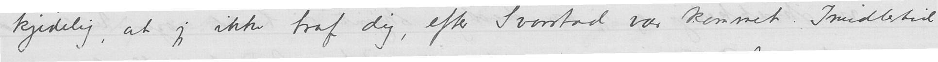kjedelig, at jeg ikke traf dig, efter Svarstad var kommet. Imidlertid
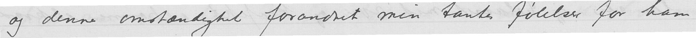og denne omstændighet forandret min tantes følelser for ham
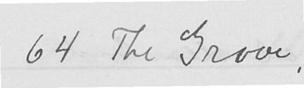64 The Grove,
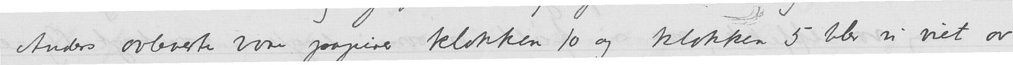Anders avleverte vore papirer klokken 10 og klokken 5 blev vi viet av
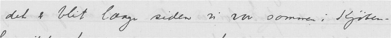det er blit længe siden vi var sammen i Kjøben-
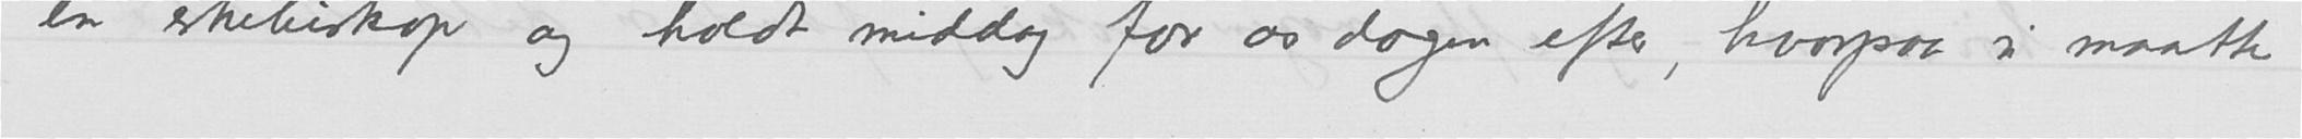en erkebiskop og holdt middag for os dagen efter, hvorpaa vi maatte
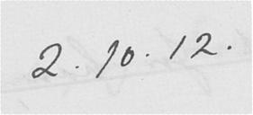2.10.12.
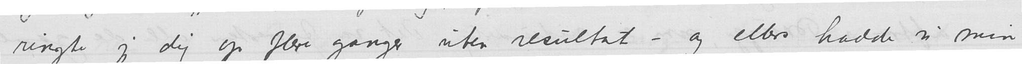ringte jeg dig op flere ganger uten resultat - og ellers hadde vi min
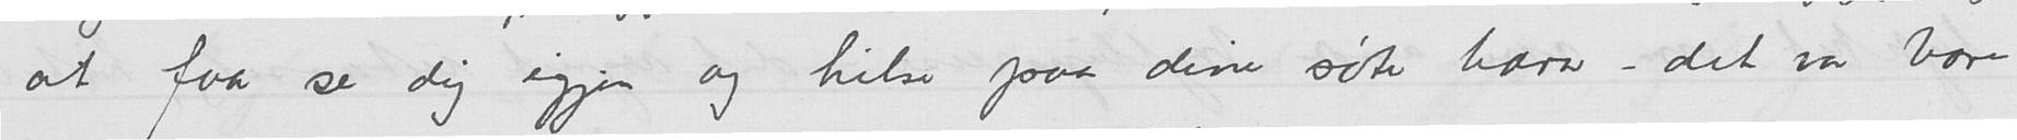at faa se dig igjen og hilse paa dine søte barn - det var bare
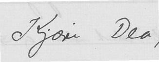Kjære Dea,
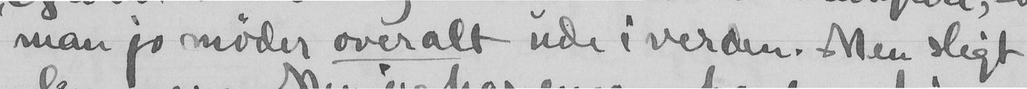man jo møder overalt ude i verden. Men sligt
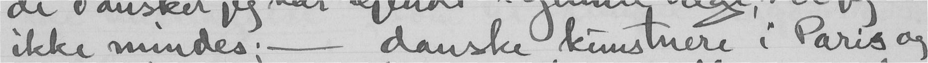ikke mindes; - danske kunstnere i Paris og
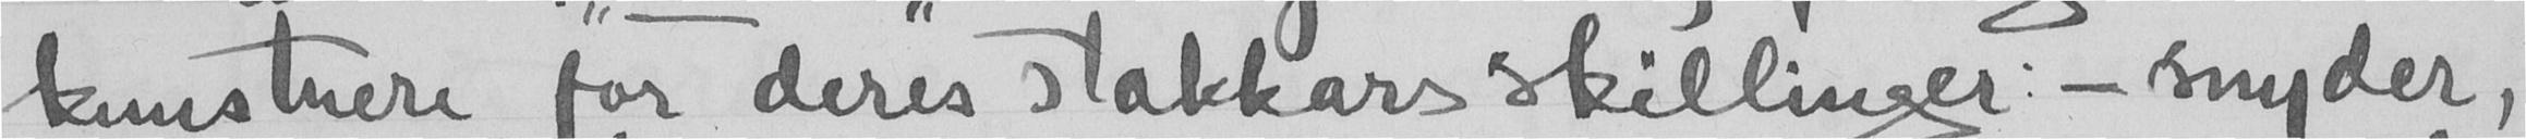kunstnere for deres stakkars skillinger: - snyder,
